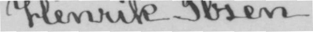Henrik Ibsen.
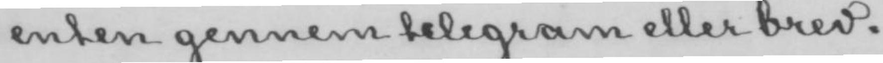enten gennem telegram eller brev.
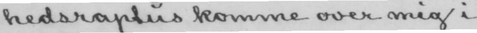hedsraptus komme over mig i
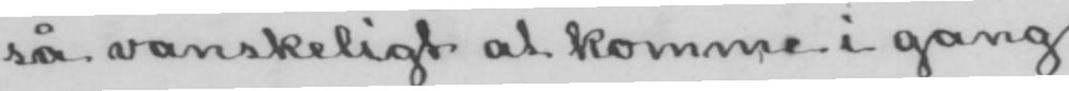så vanskeligt at komme i gang
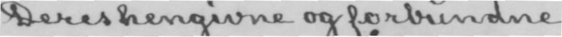Deres hengivne og forbundne
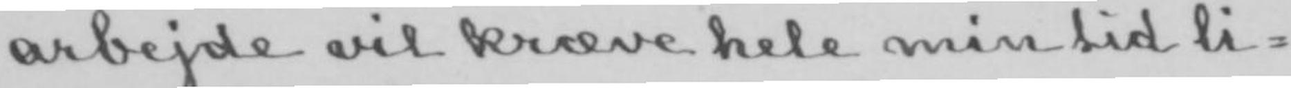arbejde vil kræve hele min tid li-
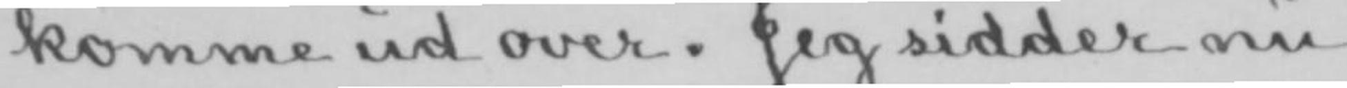komme ud over. Jeg sidder nu
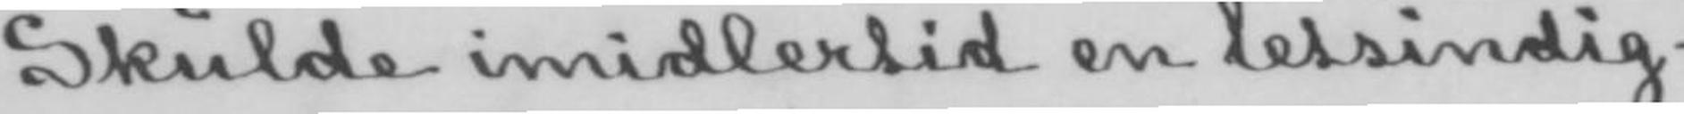Skulde imidlertid en letsindig-
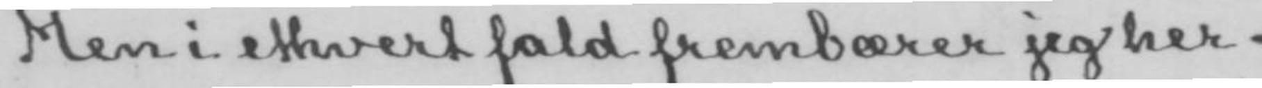Men i ethvert fald frembærer jeg her-
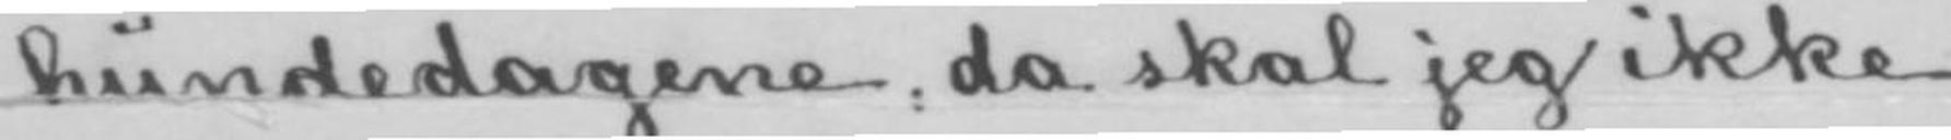hundedagene, da skal jeg ikke
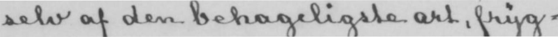selv af den behageligste art, fryg-
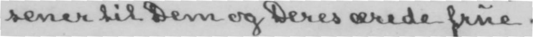sener til Dem og Deres ærede frue.
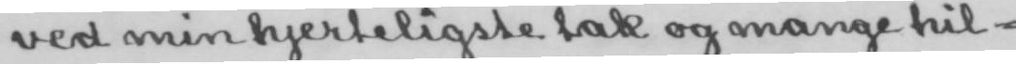ved min hjerteligste tak og mange hil-
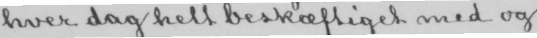hver dag helt beskæftiget med og
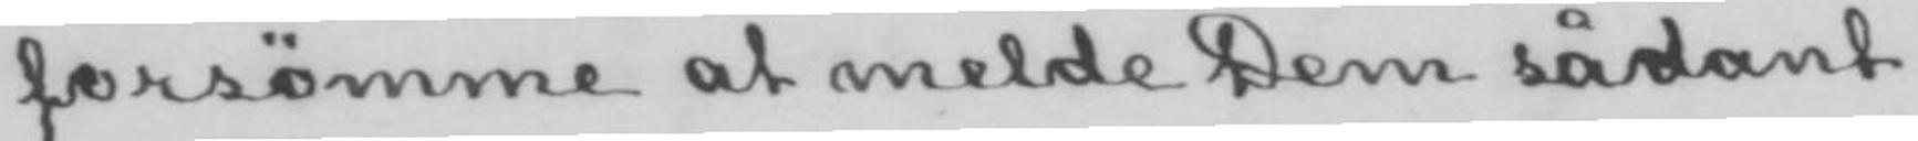forsømme at melde Dem sådant
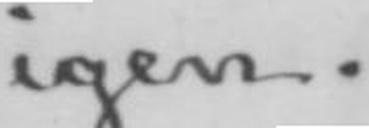igen.
